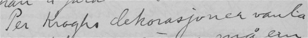Per Kroghs dekorasjoner vanla
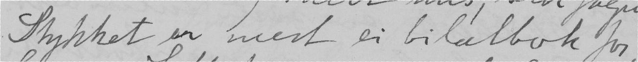Stykket er mest ei bilætbok for
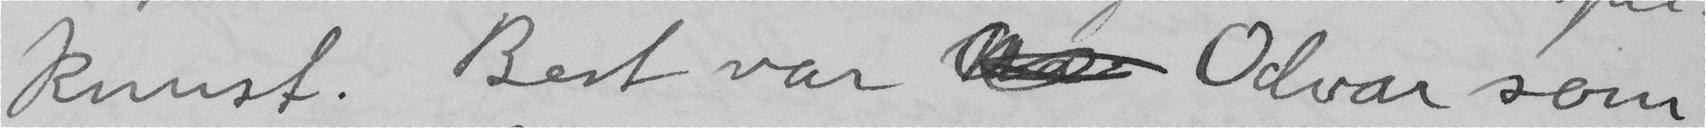kunst. Best var  Odvar som
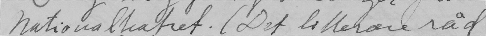Nationalteatret. (Det litterære råd
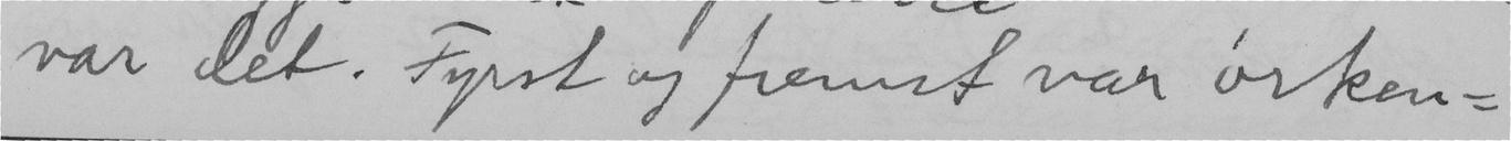var det. Fyrst og fremst var örken-
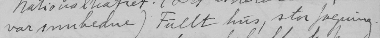var innbedne) Fullt hus, stor fagning.
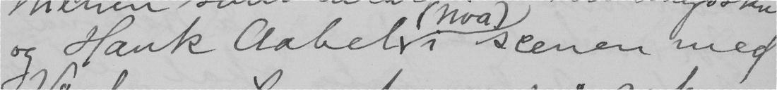og Hank Aabel i scenen med
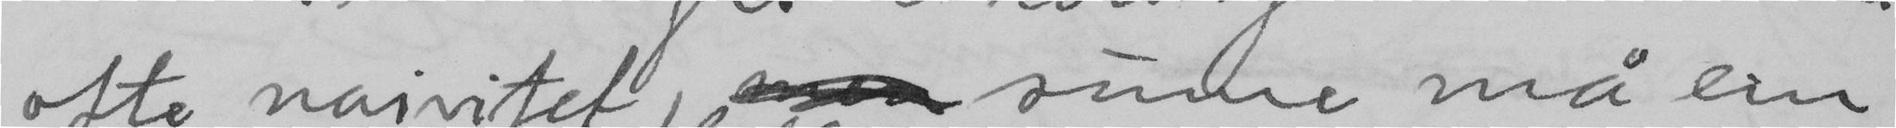ofte naivitet; sume må ein
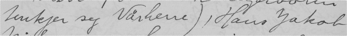tenkjer sig Vårherre), Hans Jakob
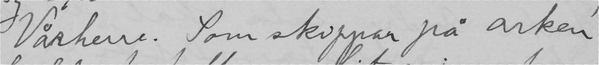Vårherre. Som skippar på arken
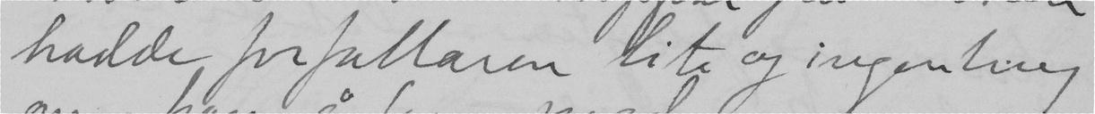hadde forfattaren lite og ingenting
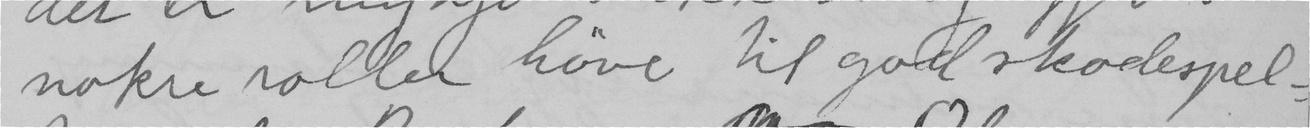nokre roller höve til god skodespel-
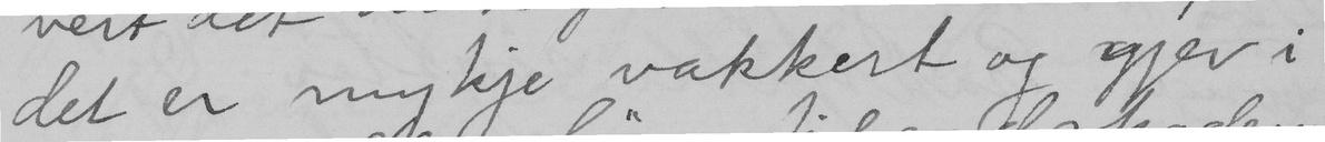det er mykje vakkert og gjev i
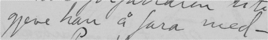gjeve han å fara med –
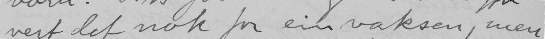vert det nok for ein vaksen, men
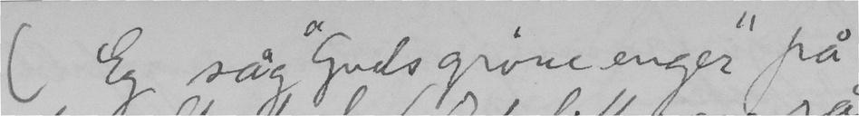(Eg såg "Guds gröne enger" på
